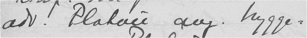adv. Platou ang. brygge-
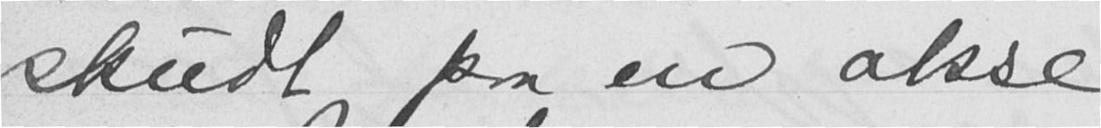skudt paa en okse
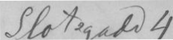Slotsgade 4.
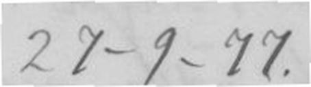27-9-77.
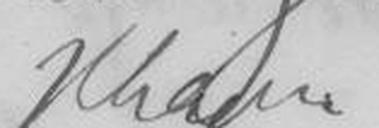Khavn
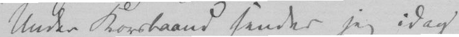Under Korsbaand finder jeg idag
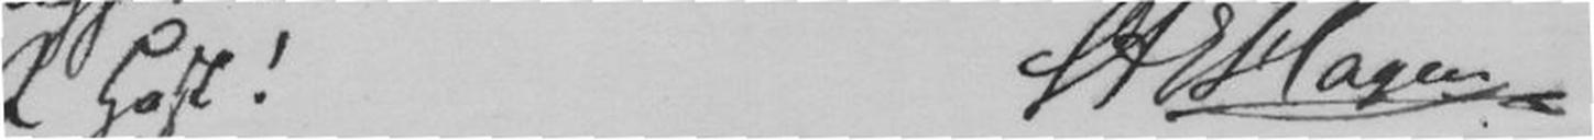I Hast! Deres hengivne S A E Hagen
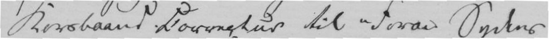Korsbaand Correctur til "Foran Sydens
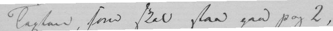Texten, som skal staa paa pog 2,
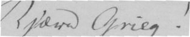Kjære Grieg!
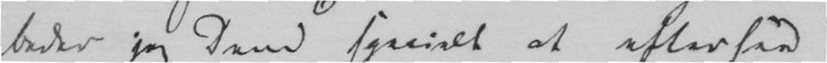beder jeg Dem specielt at eftersee.
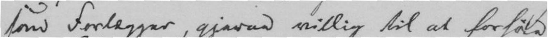som Forlægger, gjerne villig til at forhøre
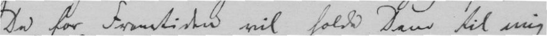De for Fremtiden vil holde Dem til mig
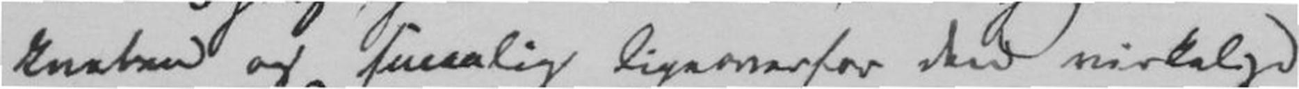Kneben og smaalig ligeoverfor den virkelige
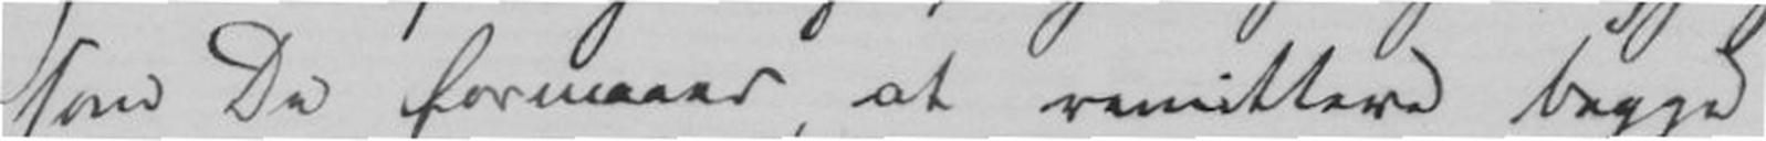som De formaaer, at remittere begge
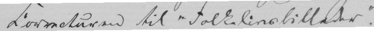Correcturen til "Folkelivsbilleder".
